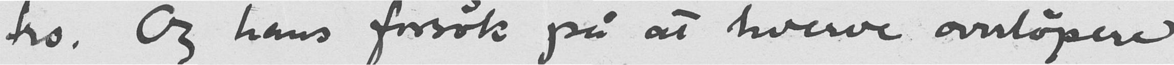tro. Og hans forsøk på at hverve overløpere
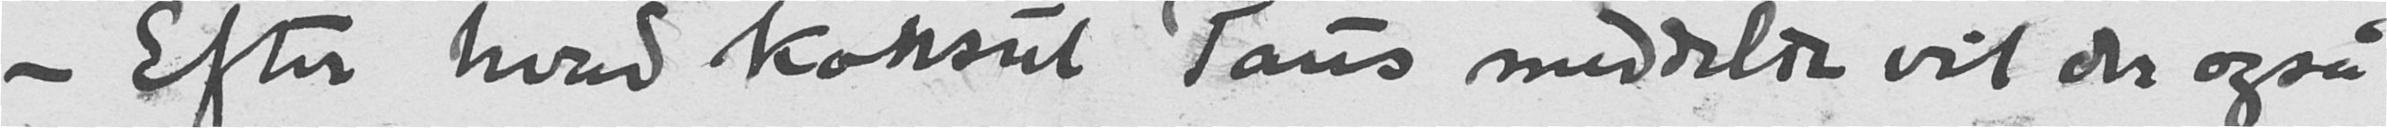- Efter hvad konsul Paus meddeler vil der også
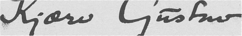Kjære Gustav
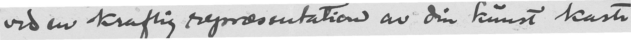ved en kraftig repræsentation av din kunst kaste
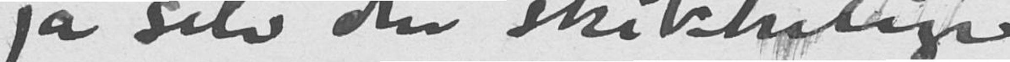ja selv den skikkelige
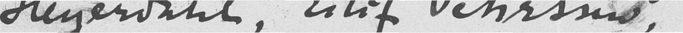Heyerdahl, Eilif Petersen,
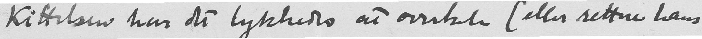Kittelsen har det lykkedes at overtale (eller rettere hans
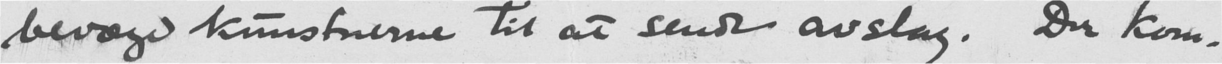bevæge kunstnerne til at sende avslag. Der kom-
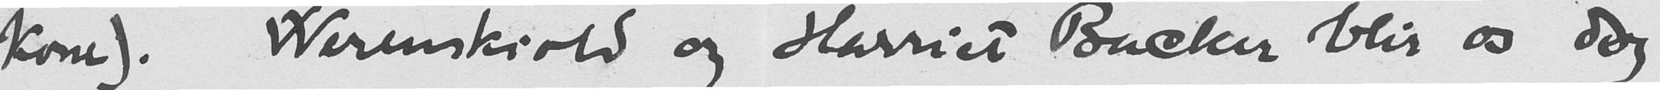kone). Werenskiold og Harriet Backer blir os dog
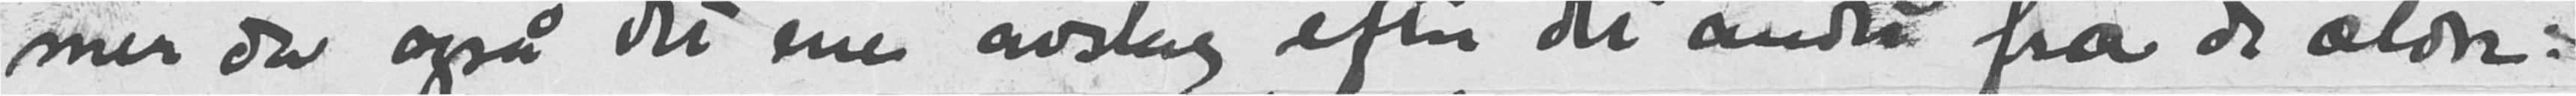mer da også det ene avslag efter det andet fra de ældre:
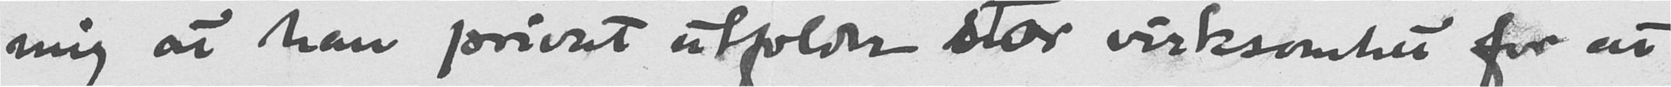mig at han privat utfolder stor virksomhet for at
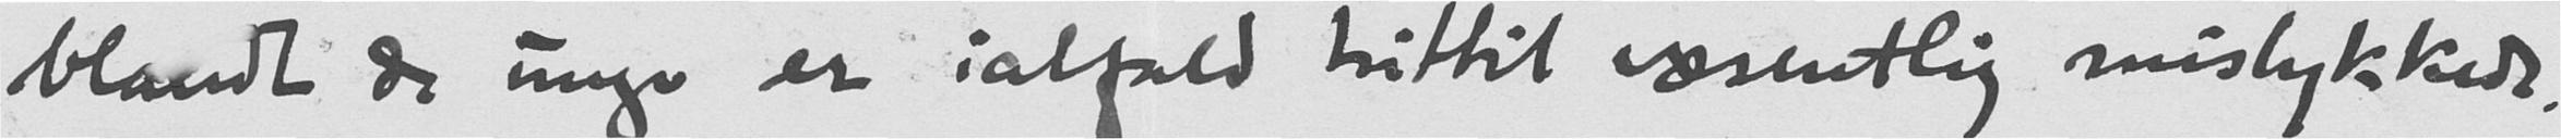blandt de unge er ialfald hittil væsentlig mislykkede.
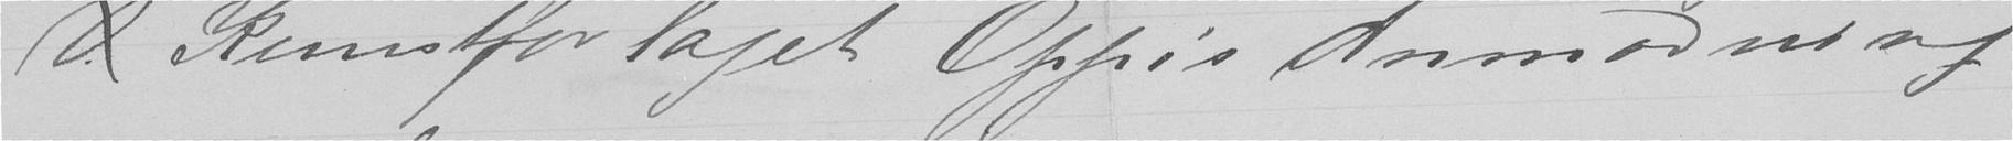D. Kunstforlaget Oppis Anmodning
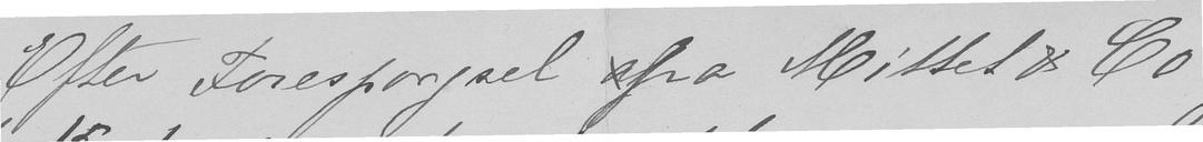Efter Forespørgsel afra Mittet & Co
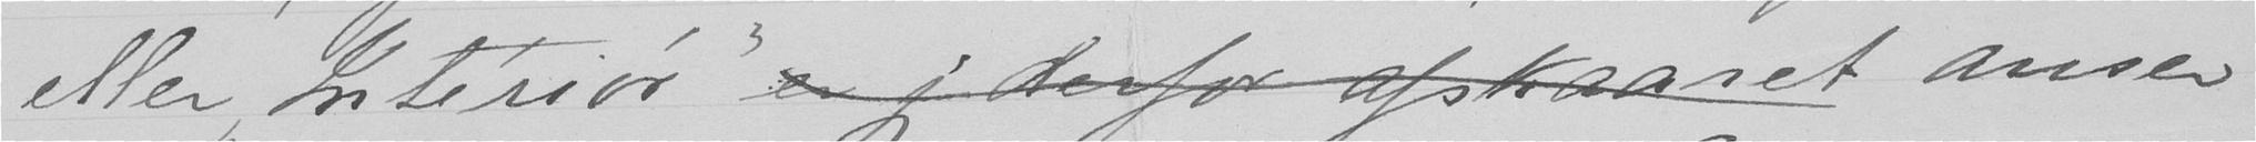eller Interiør" er jeg derfor afskaaret anser
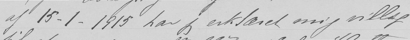af 15-1-1915 har jeg erklæret mig villig
